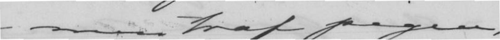- men traf pigen,
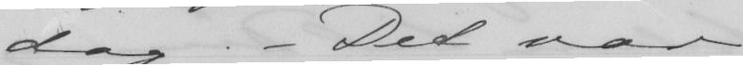dag. - Det var
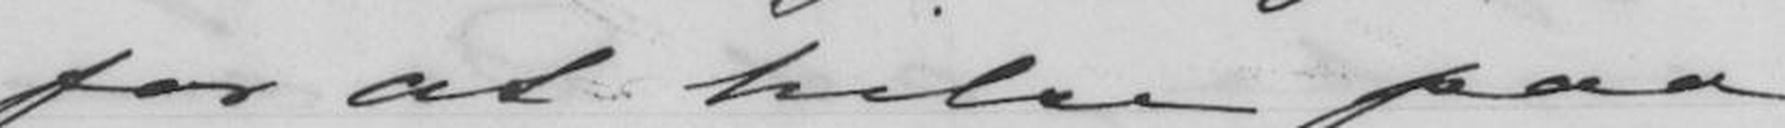for at hilse paa
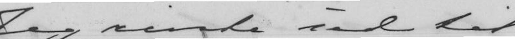Jeg reisde ud til
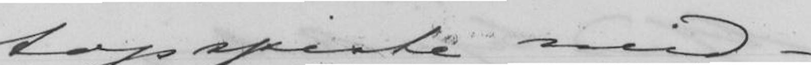top spiste mid-
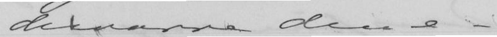desværre den e-
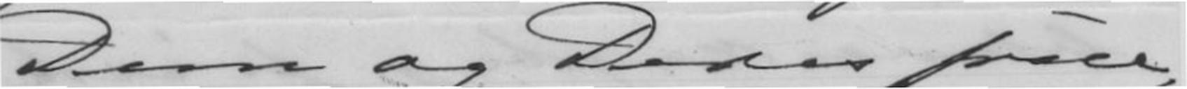Dem og Deres frue,
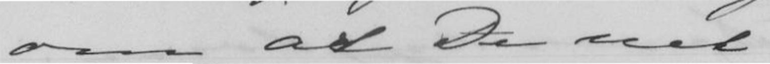om, at De net-
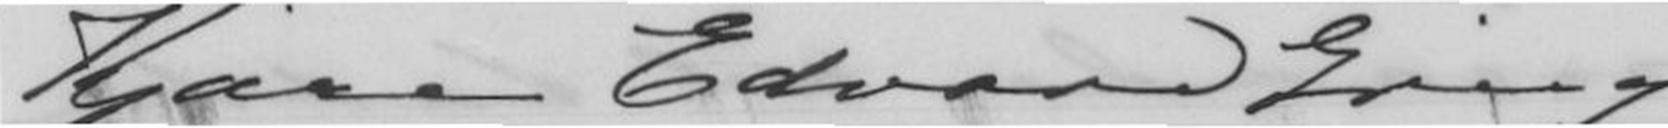Kjære Edvard Grieg!
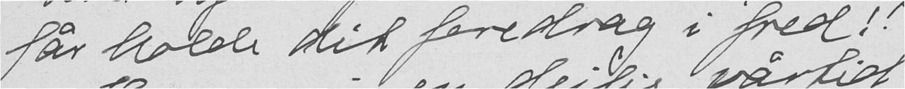får holde dit foredrag i fred!!
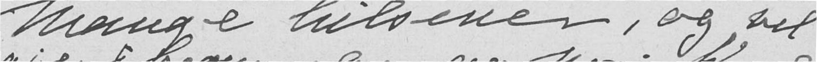Mange hilsener, og vel
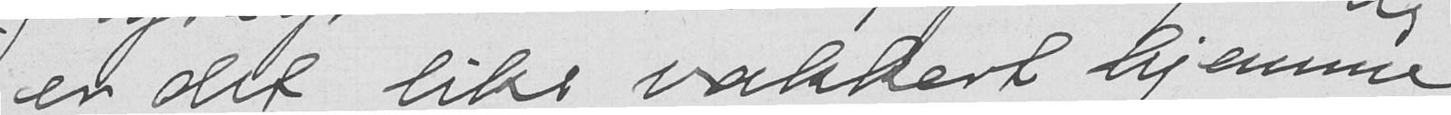er det like vakkert hjemme
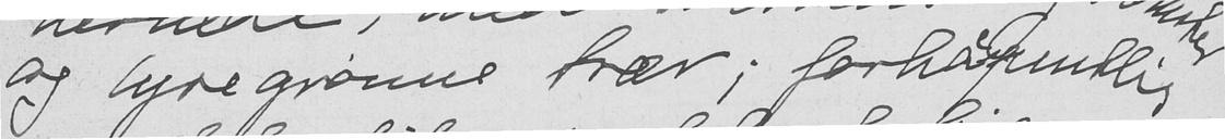og lysegrønne trær; forhåpentlig
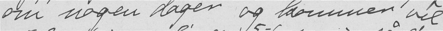om nogen dager og kommer vel
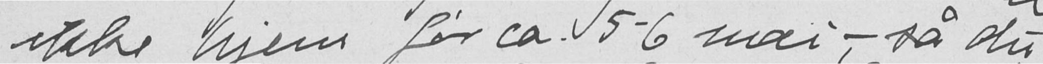ikke hjem før ca. 5-6 mai, - så du
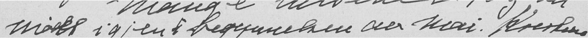mødt igjen i begynnelsen av mai. Kristine
